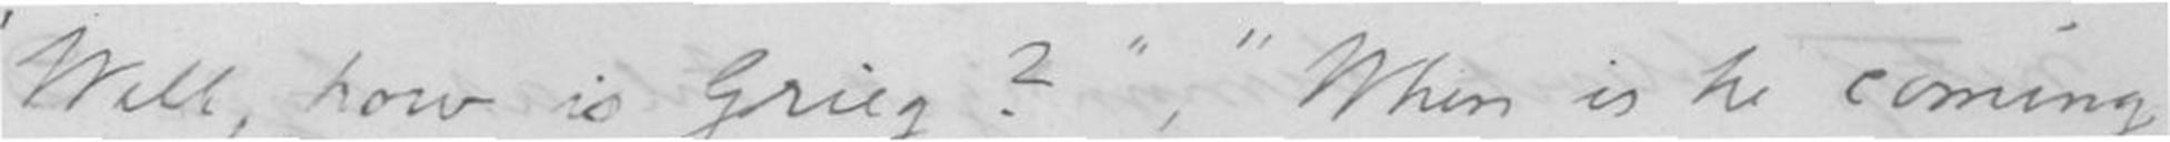"Well, how is Grieg?", "When is he coming
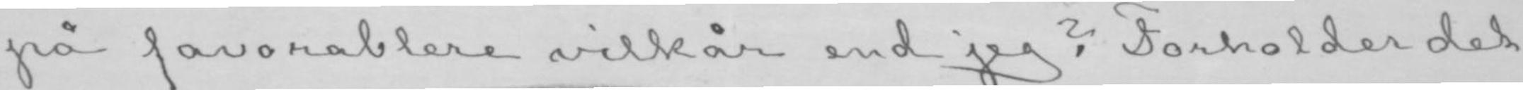på favorablere vilkår end jeg? Forholder det
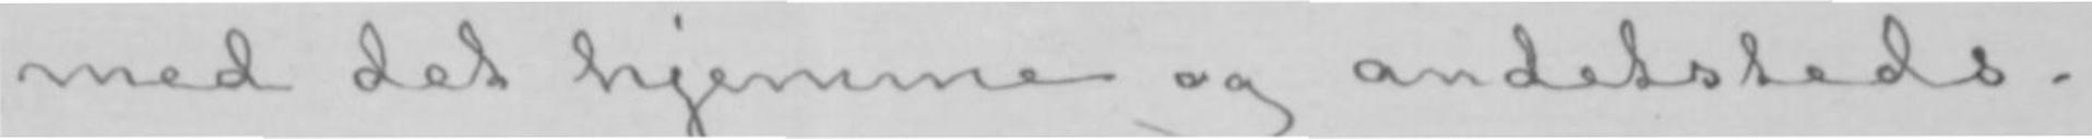med det hjemme og andetsteds.
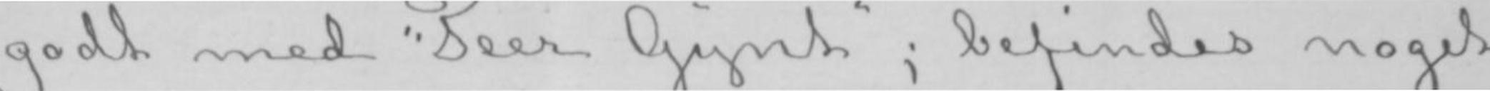godt med «Peer Gynt»; befindes noget
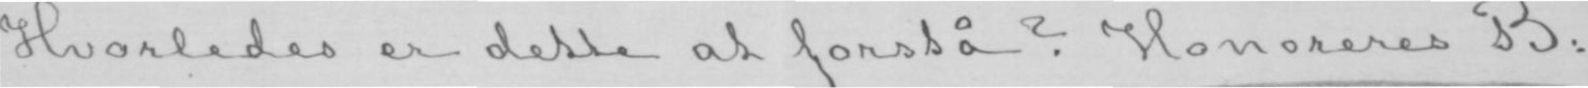Hvorledes er dette at forstå? Honoreres B:
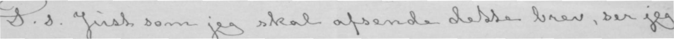P. s. Just som jeg skal afsende dette brev, ser jeg
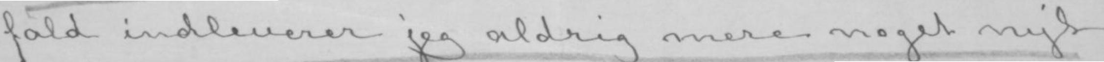fald indleverer jeg aldrig mere noget nyt
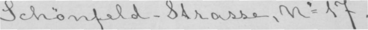Schönfeld-Strasse, No 17.
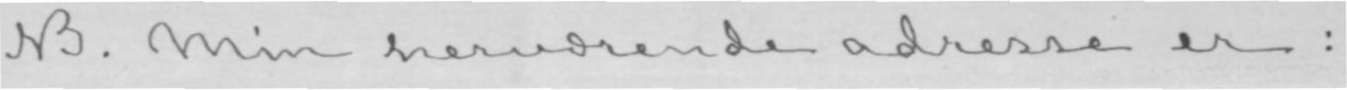NB. Min herværende adresse er:
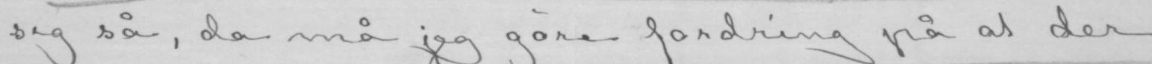sig så, da må jeg gøre fordring på at der
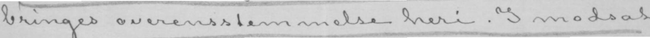bringes overensstemmelse heri. I modsat
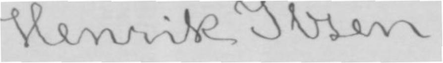Henrik Ibsen.
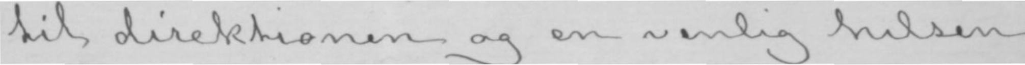til direktionen og en venlig hilsen
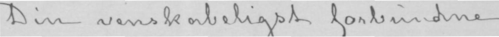Din venskabeligst forbundne
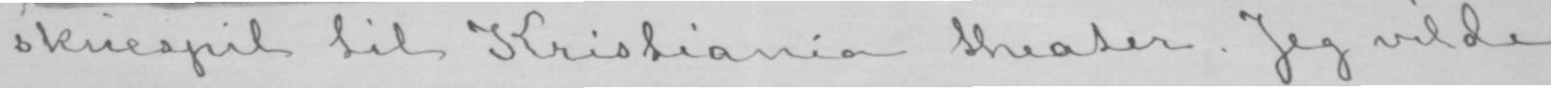skuespil til Kristiania theater. Jeg vilde
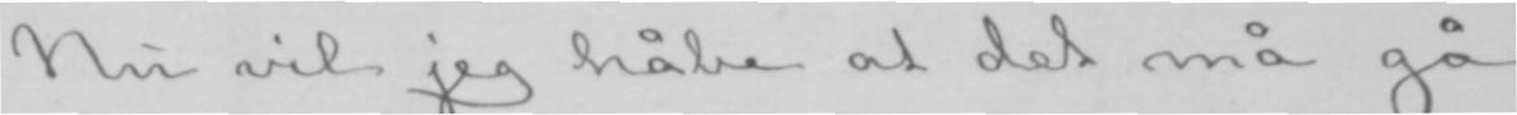Nu vil jeg håbe at det må gå
